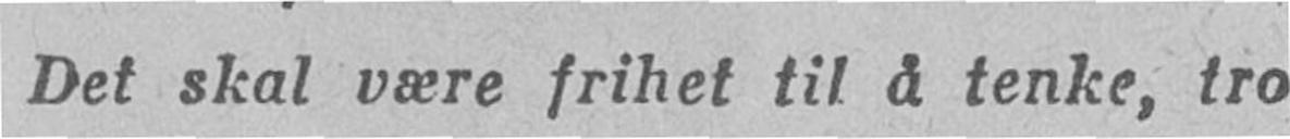Det skal være frihet til å tenke, tro
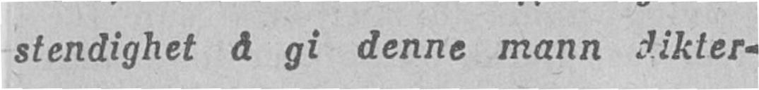stendighet å gi denne mann dikter-
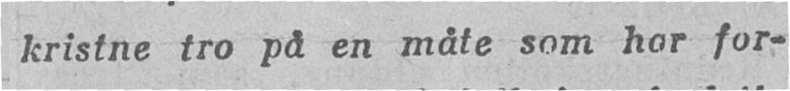kristne tro på en måte som har for-
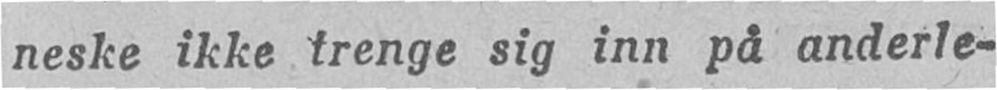neske ikke trenge sig inn på anderle-
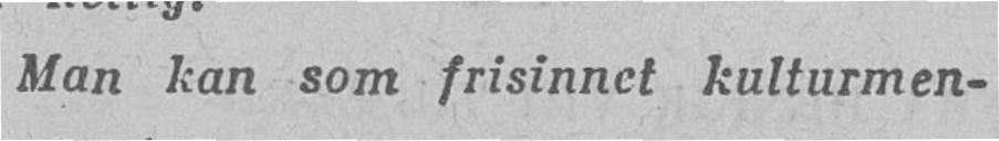Man kan som frisinnet kulturmen-
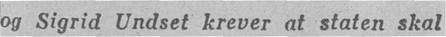og Sigrid Undset krever at staten skal
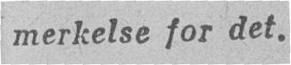merkelse for det.
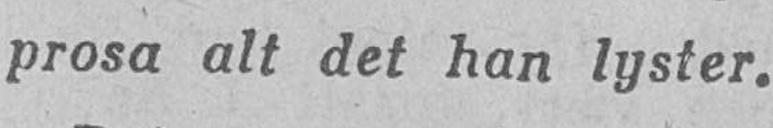prosa alt det han lyster.
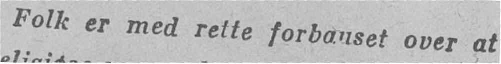Folk er med rette forbauset over at
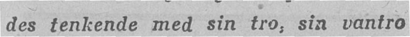des tenkende med sin tro, sin vantro
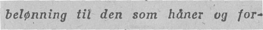belønning til den som håner og for-
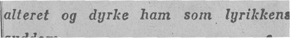alteret og dyrke ham som lyrikkens
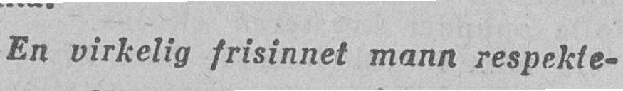En virkelig frisinnet mann respekte-
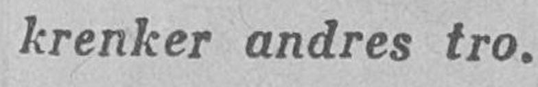krenker andres tro.
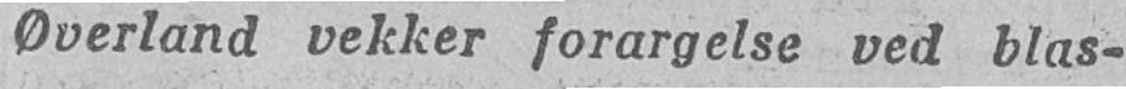Øverland vekker forargelse ved blas-
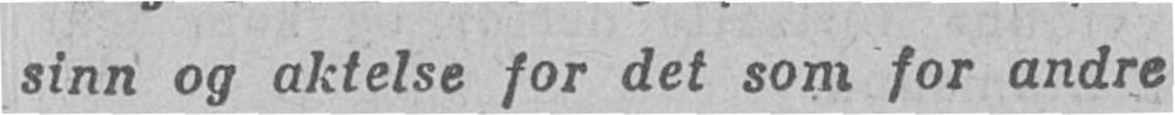sinn og aktelse for det som for andre
